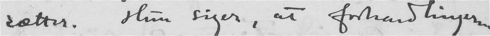sætter. Hun siger, at forhandlingerne
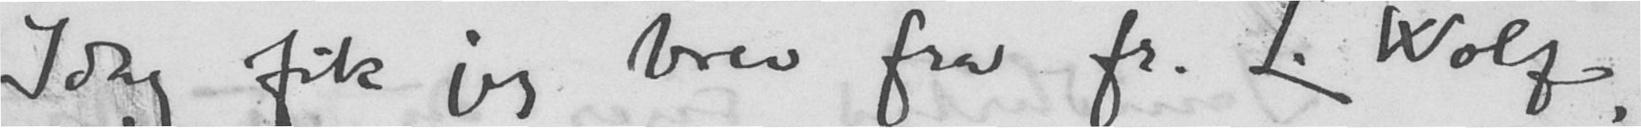Idag fik jeg brev fra fr. L. Wolf,
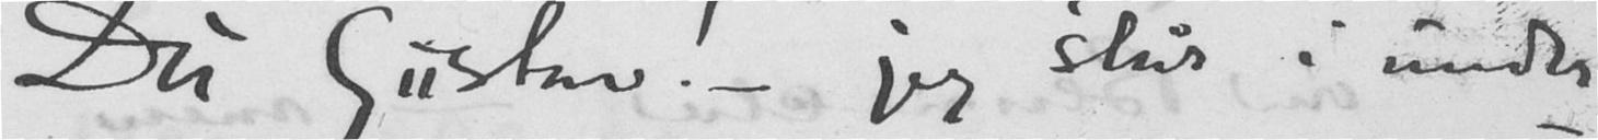Du Gustav! - Jeg står i under-
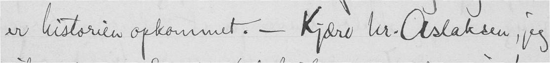er historien opkommet. - Kjære hr. Aslaksen, jeg
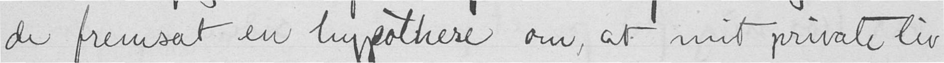de fremsat en hypothese om at mit private liv
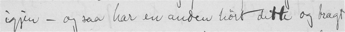igjen - og saa har en anden hørt dette og bragt
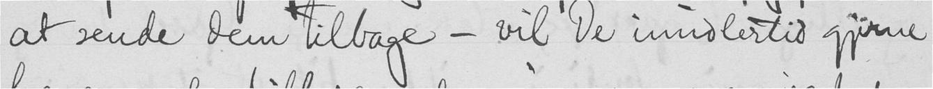at sende dem tilbage - vil De imidlertid gjerne
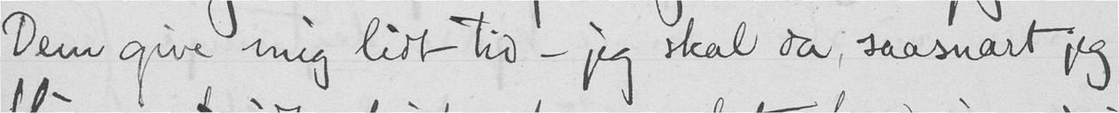Dem give mig lidt tid - jeg skal da, saasnart jeg
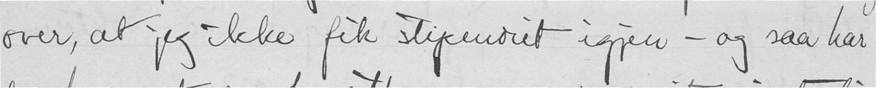over, at jeg ikke fik stipendiet igjen - og saa har
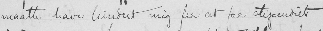maatte have hindret mig fra at faa stipendiet
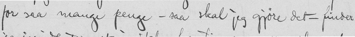for saa mange penge - saa skal jeg gjøre det - finder
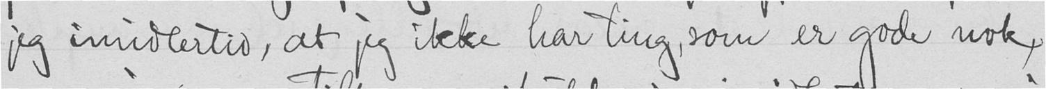jeg umidlertid, at jeg ikke har ting, som er gode nok,
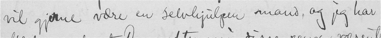vil gjerne være en selvhjulpen mand, og jeg har
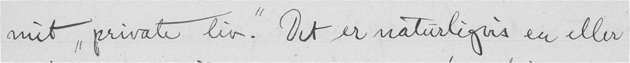mit "private liv". Det er naturligvis en eller
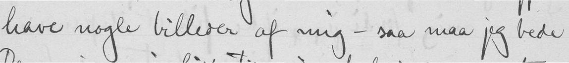have nogle billeder af mig - saa maa jeg bede
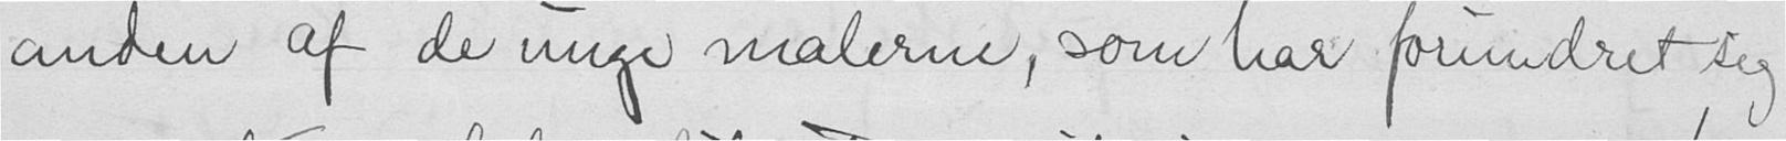anden af de unge malerne, som har forundret sig
